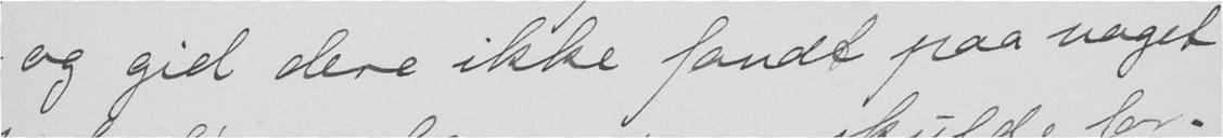og gid dere ikke fandt paa noget
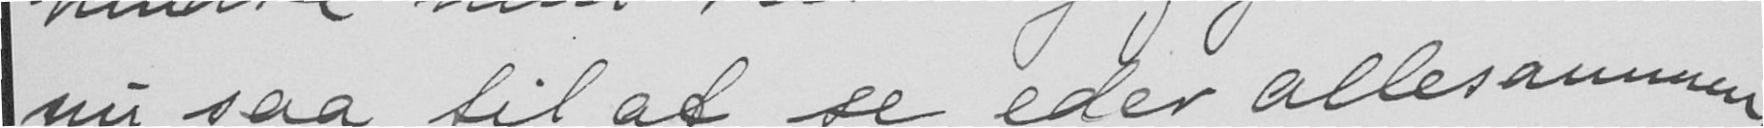nu saa til at se eder allesammen,
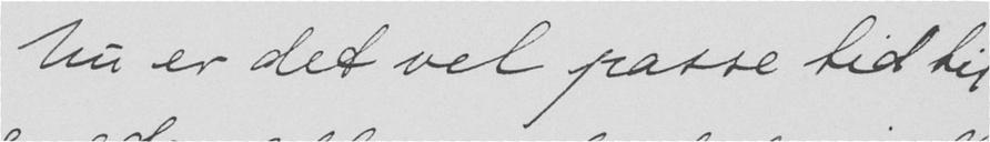Nu er det vel passe tid til
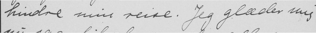hindre min reise. Jeg glæder mig
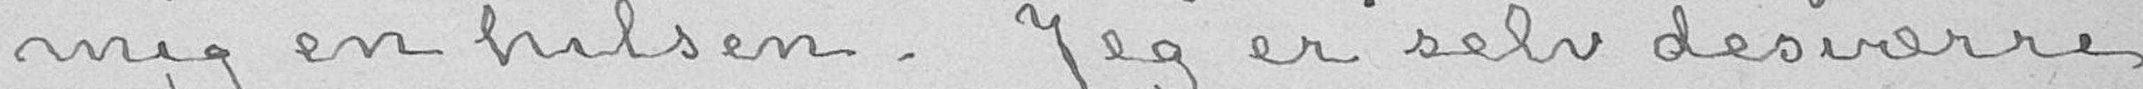mig en hilsen. Jeg er selv desværre
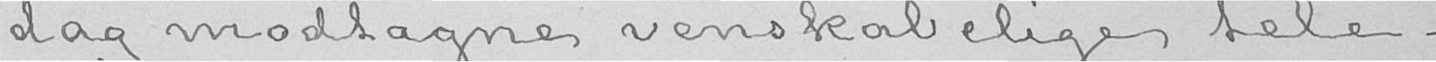dag modtagne venskabelige tele-
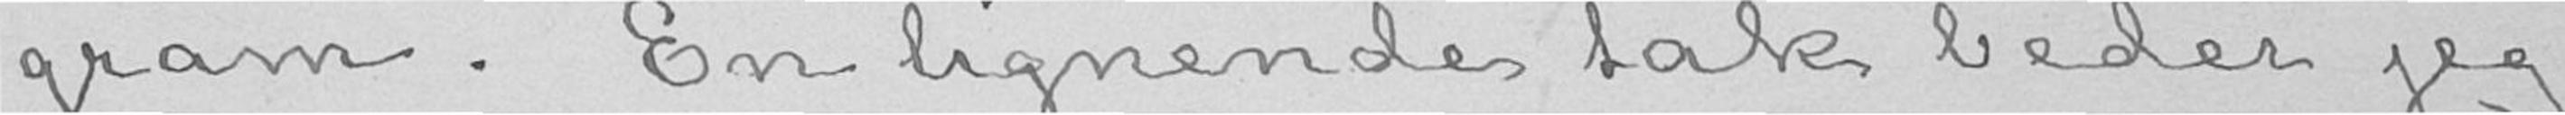gram. En lignende tak beder jeg
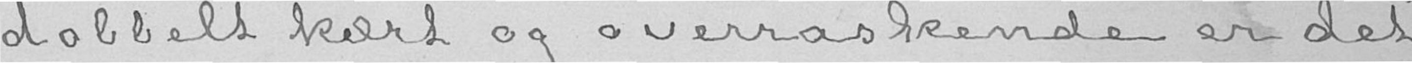dobbelt kært og overraskende er det
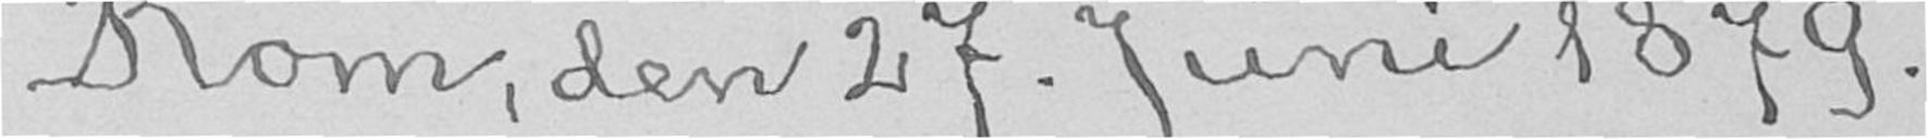Rom, den 27. Juni 1879.
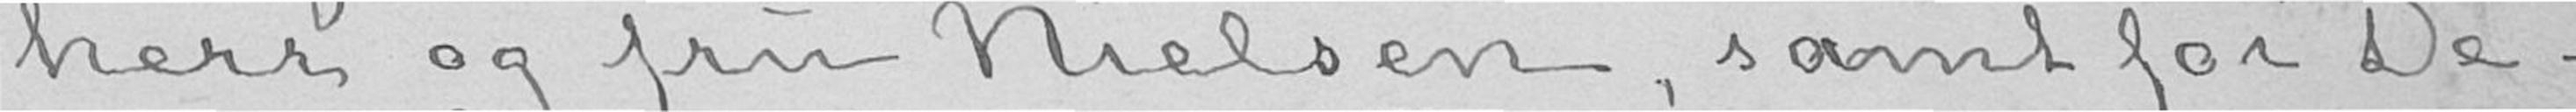herr og fru Nielsen, samt for De-
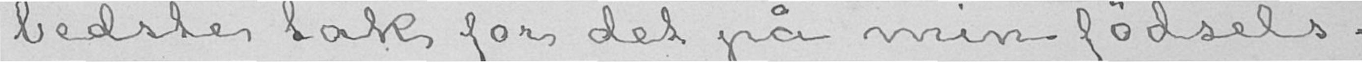beste tak for det på min fødsels-
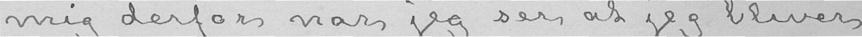mig derfor når jeg ser at jeg bliver
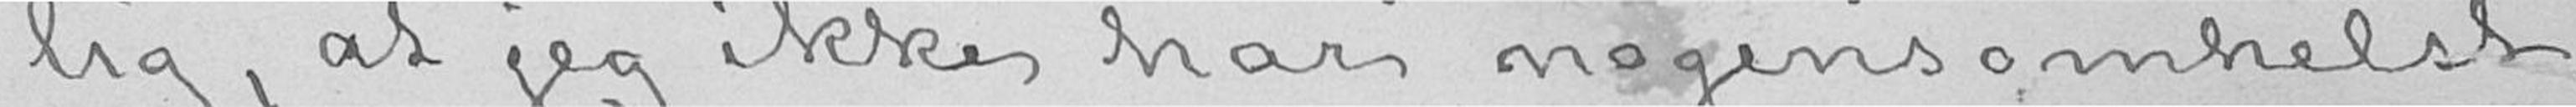lig, at jeg ikke har nogensomhelst
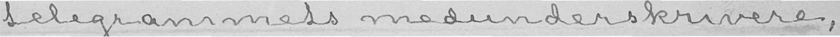telegrammets medunderskrivere,
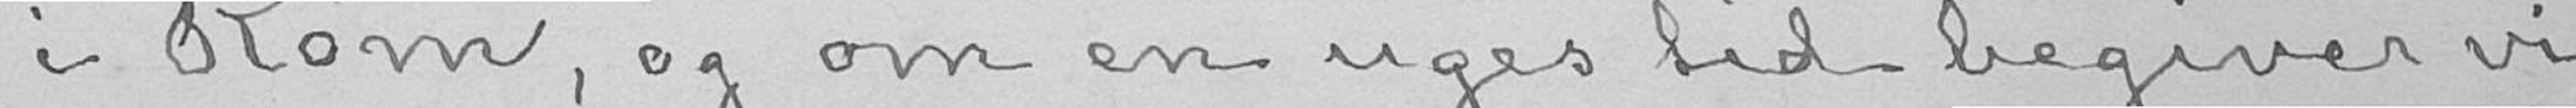i Rom, og om en uges tid begiver vi
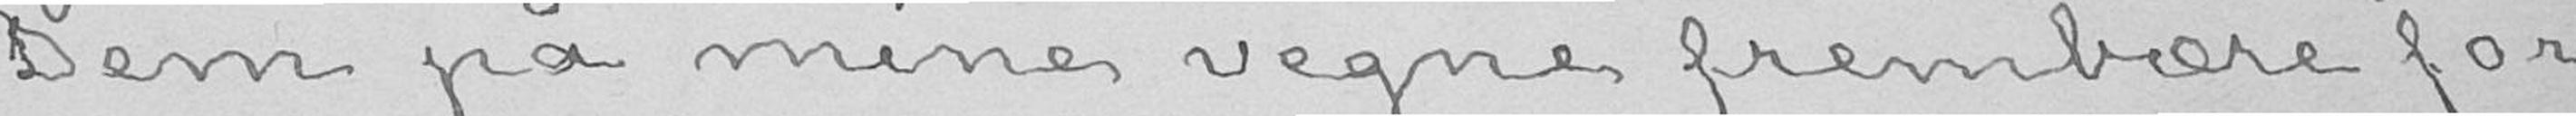Dem på mine vegne frembære for
